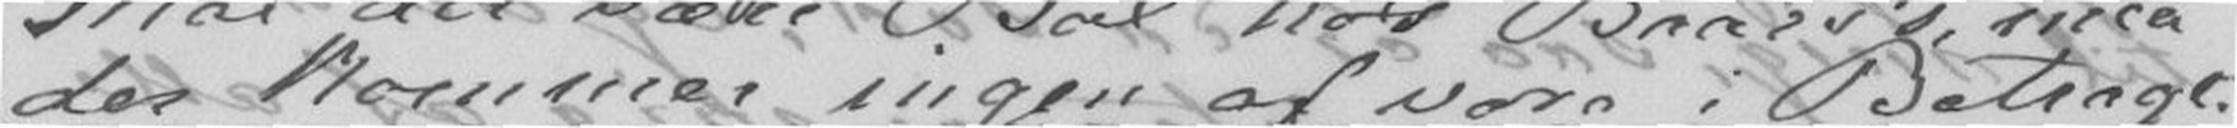der kommer ingen af vore i Betragt-
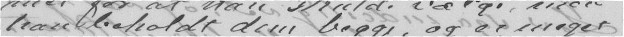han beholdt dem begge, og er meget
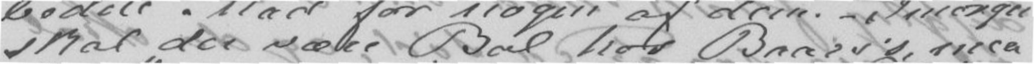skal der være Bal hos Baars's, men
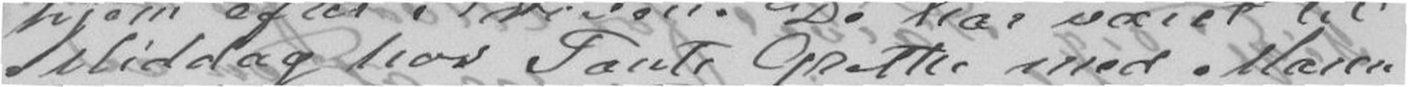Middag hos Tante Grethe med Maren
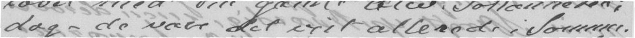dog - de vare det vist allerede i Sommer.
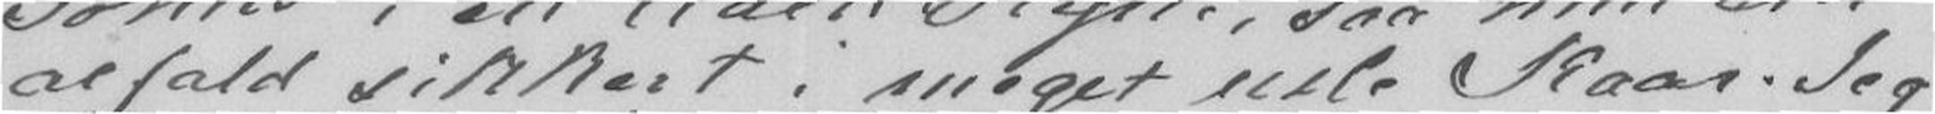alfald sikkert i meget usle Kaar. Jeg
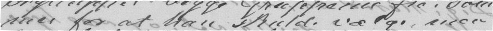mer for at han skulde vælge, men
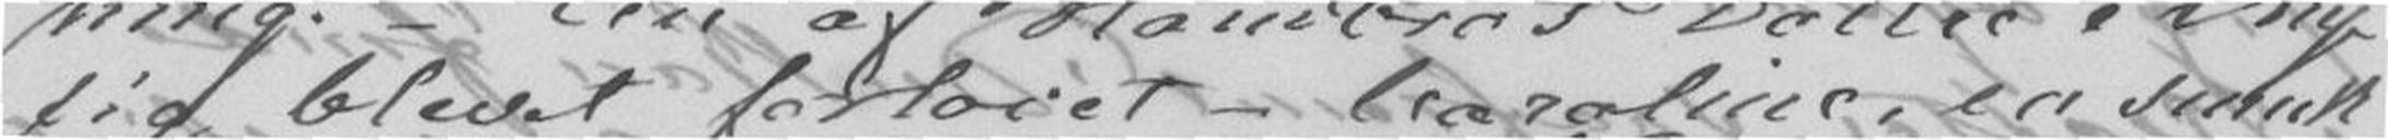lig blevet forlovet - Caroline, en smuk
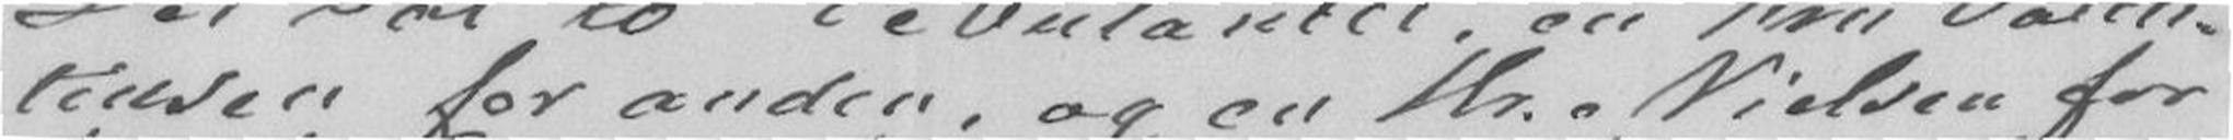tinsen for anden, og en Hr. Nielsen for
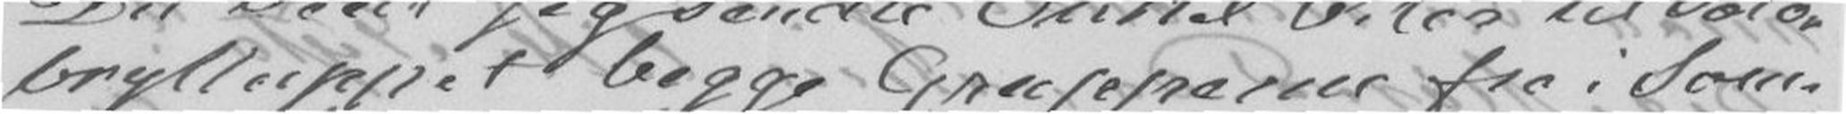brylluppet begge Grupperne fra i Som-
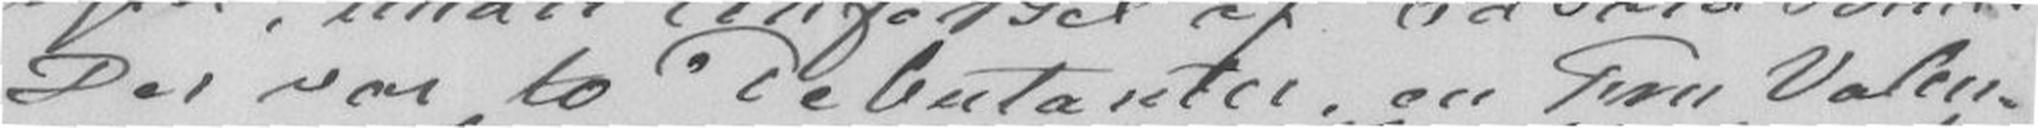Der var to Debutanter, en Fru Valen-
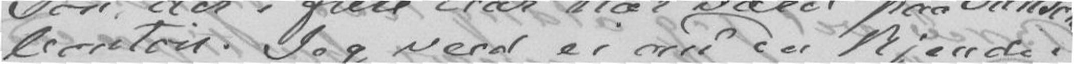Contoir. Jeg veed ei om Du kjende
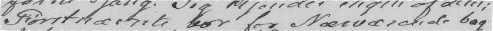Førstnævnte bor for Nærværende bag
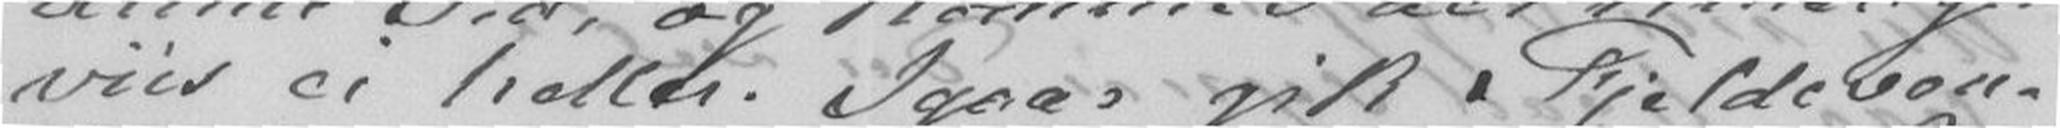viis ei heller. Igaar gik Fjeldeven-
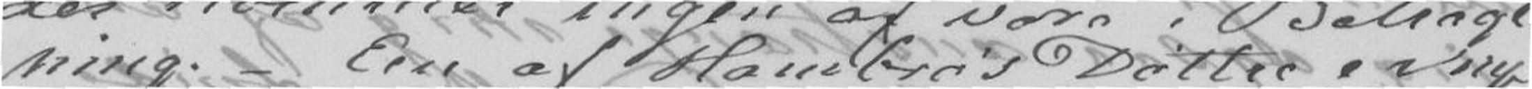ning. - En af Hambro's Døttre er ny-
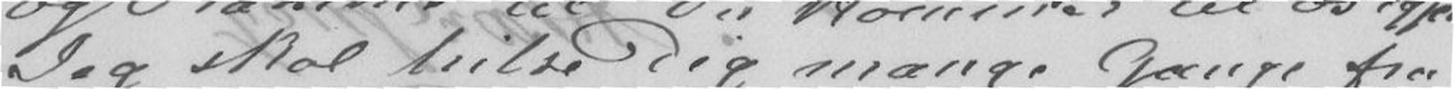Jeg skal hilse Dig mange Gange fra
